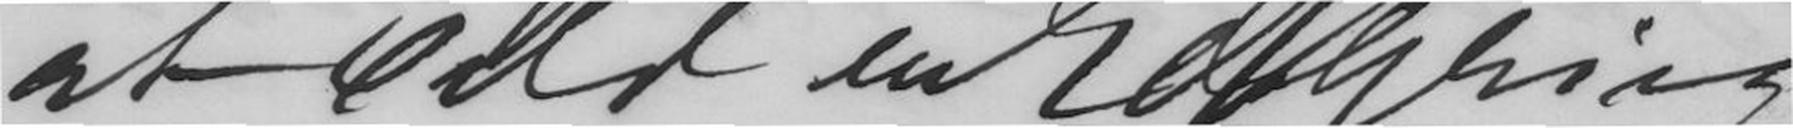at selv en Edv Grieg
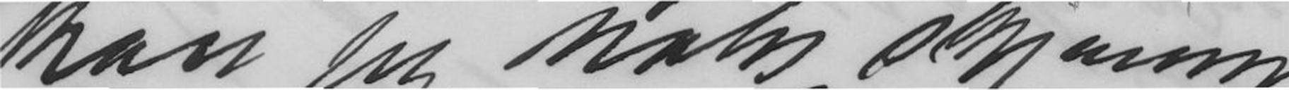kan jeg nok skjønne
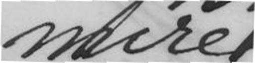mere
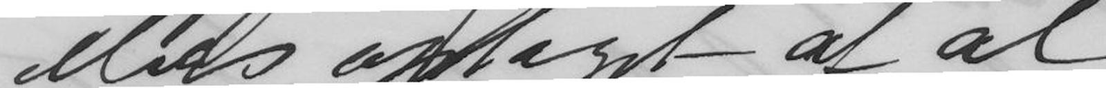ellers Optaget af al
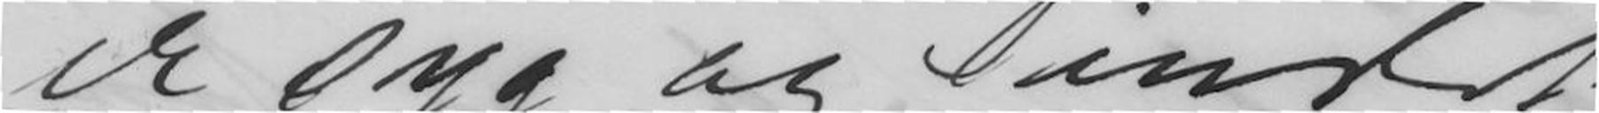er syg og Sindet
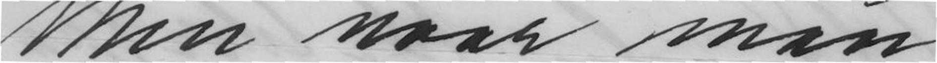Men naar man
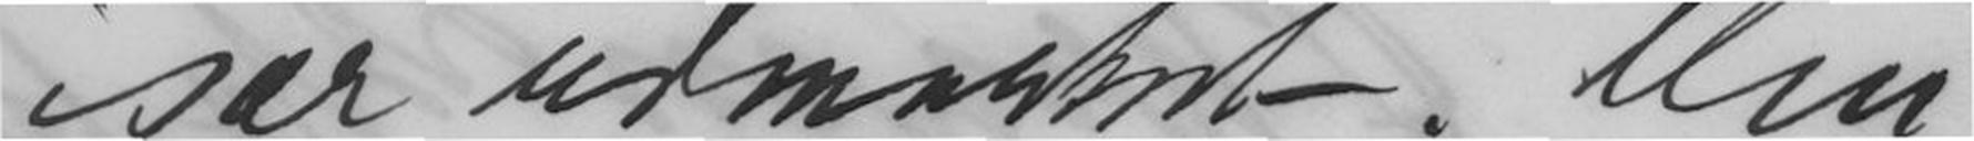især udmærket. Men
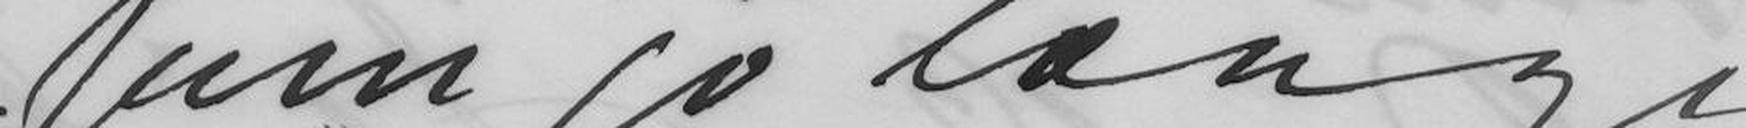som jo længe
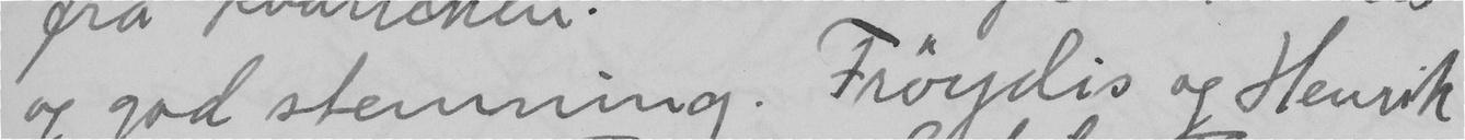og god stemning. Fröydis og Henrik
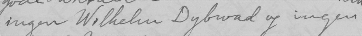ingen Wilhelm Dybwad og ingen
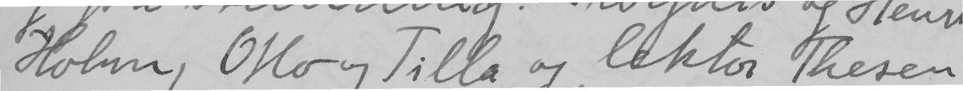Holm, Otto og Tilla og lektor Thesen
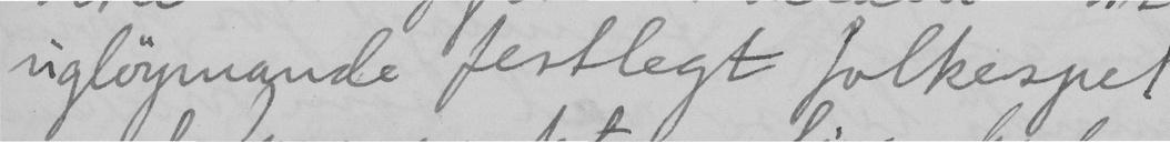uglöymande festlegt folkespel
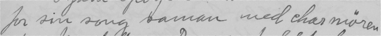for sin song saman med Charmören
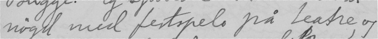nögd med festspelo på teatre, og
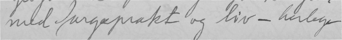med Jurgeprakt og liv – herlege
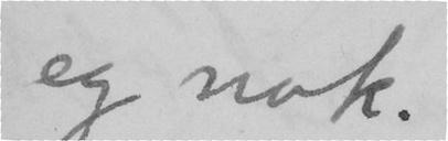eg nok.
